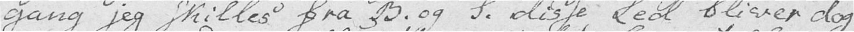gang jeg skilles fra B. og S. disse Led bliver dog
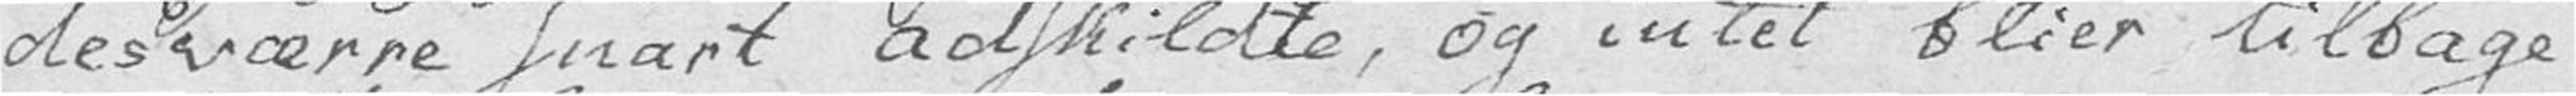desværre snart adskildte, og intet blier tilbage
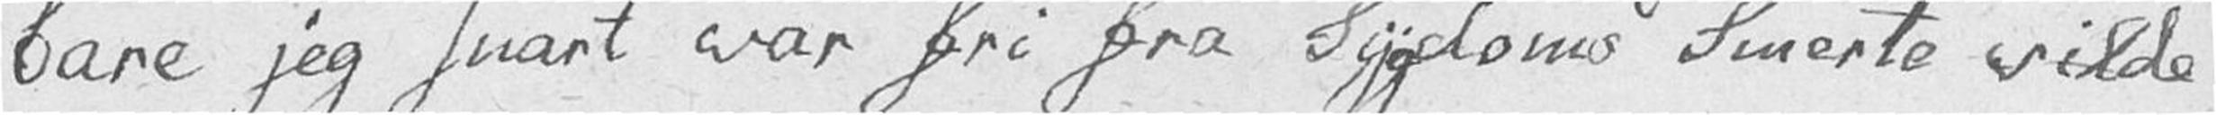bare jeg snart var fri fra Sykdoms Smerte vilde
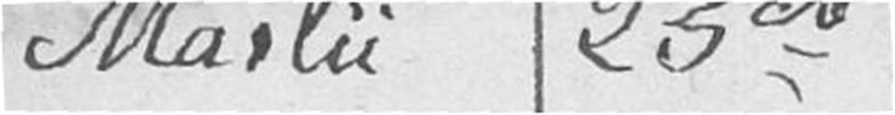Martii 23de
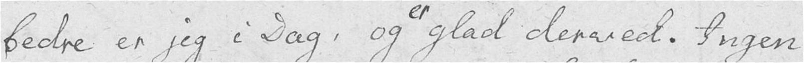bedre er jeg i Dag, og er glad derved. Ingen
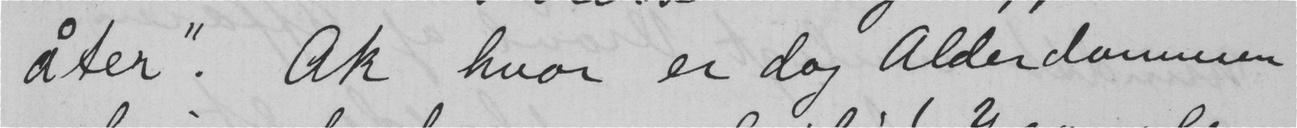åter". Ak hvor er dog alderdommen
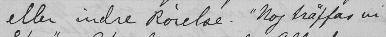eller indre Rørelse. "Nog träffas vi
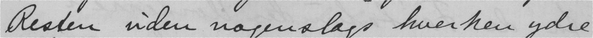Resten uden nogenslags hverken ydre
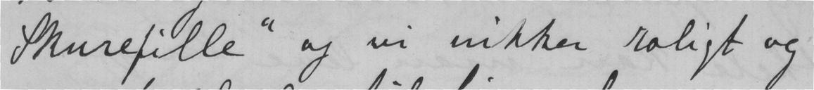Skurefille" og vi nikker roligt og
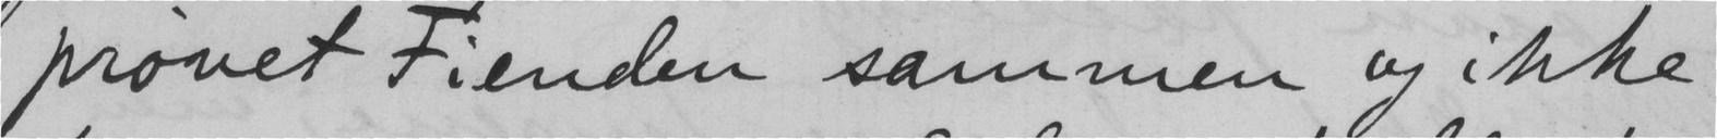provet Fienden sammen og ikke
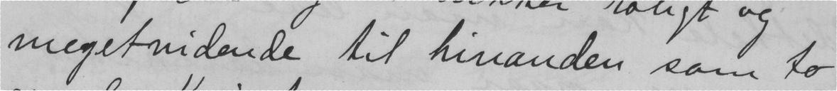megetvidende til hinanden som to
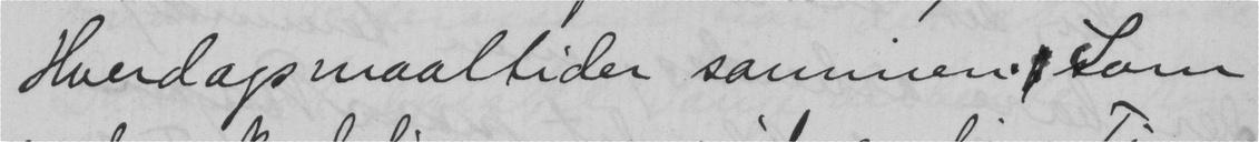Hverdagsmaaltider sammen som
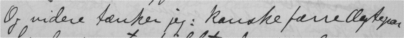Og videre tænker jeg: kanske færre Ægtepar
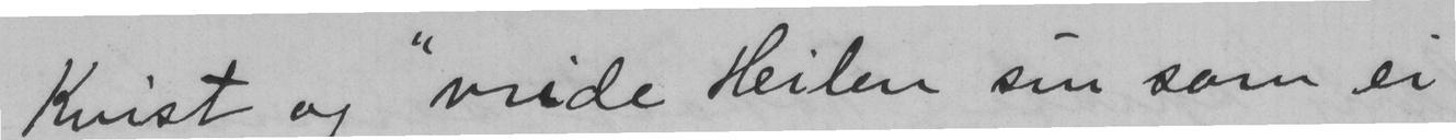Kvist og "vride Heilen sin som ei
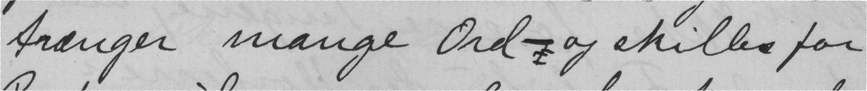trænger mange Ord - og skilles for
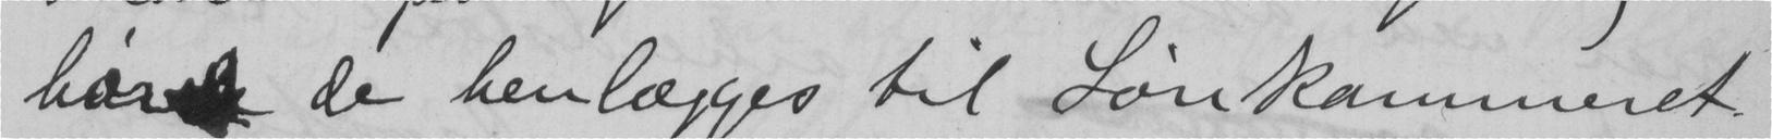bør de henlægges til Lønkammeret.
